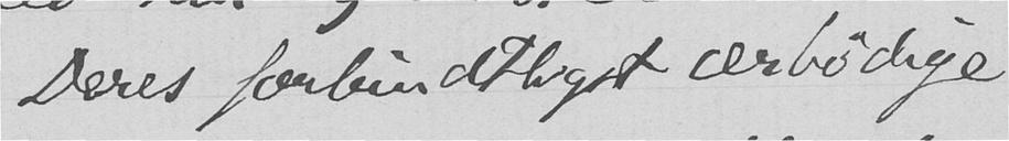Deres forbindtligst ærbødige
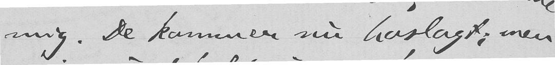mig. De kommer nu hoslagt; men
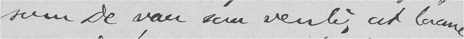som De var saa venlig at laane
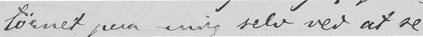tørnet paa mig selv ved at se,
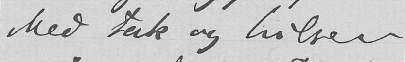Med tak og hilsen
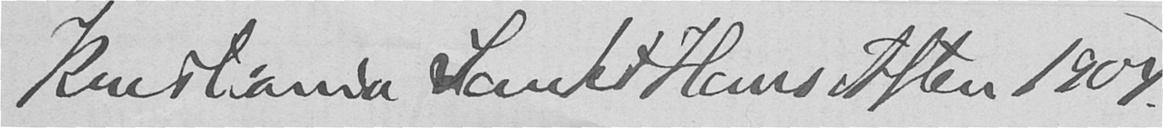Kristiania Sankt Hans Aften 1904.
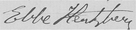Ebbe Hertzberg.
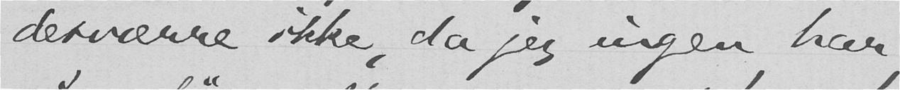desværre ikke, da jeg ingen har
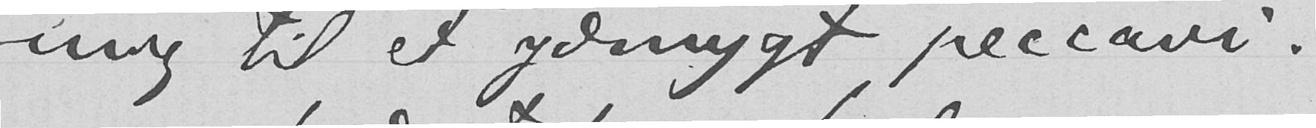mig til et ydmygt peccavi.
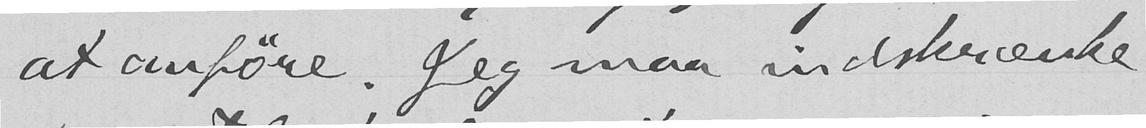at anføre. Jeg maa indskrænke
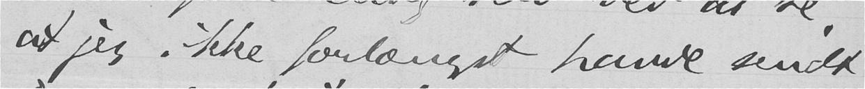at jeg ikke forlængst havde sendt
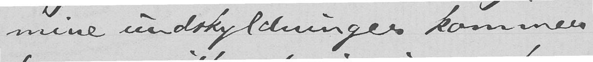mine undskyldninger kommer
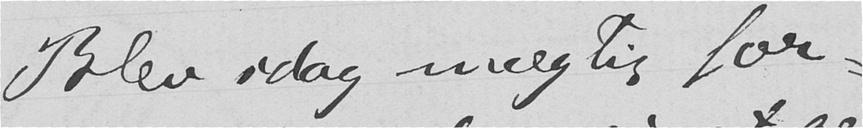Blev idag mægtig for-
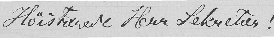Høistærede Herr Sekretær!
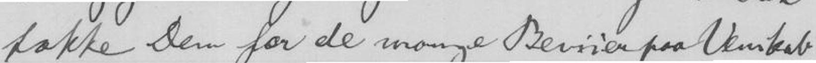takke Dem for de mange Bevisen paa Venskab,
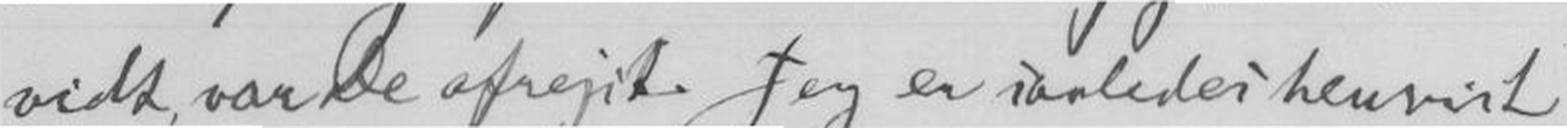vidt, var De afrejst. Jeg er saaledes henvist
